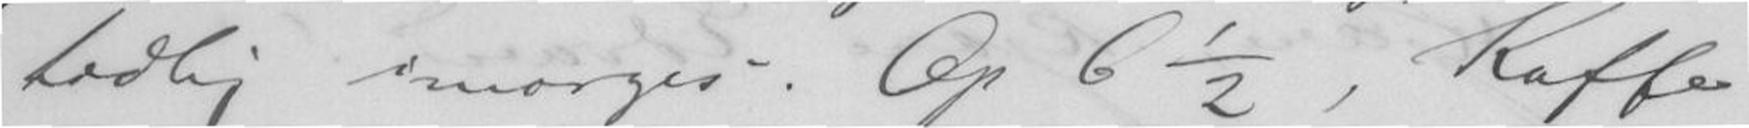tidlig imorges. Op 6 ½, Kaffe
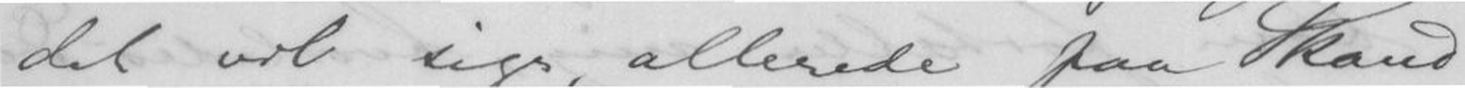det vil sige, allerede paa Skand-
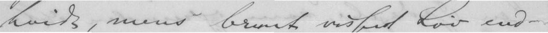hvidt, mens brunt visset Løv end-
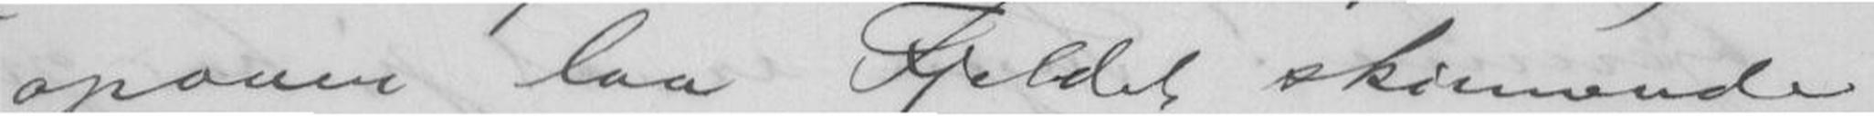opover laa Fjeldet skinnende
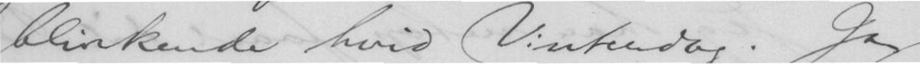blinkende hvid Vinterdag. Ja
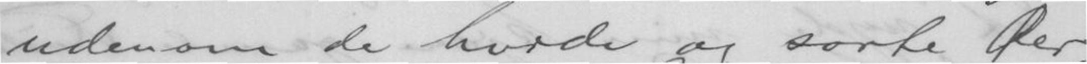udenom de hvide og sorte Øer,
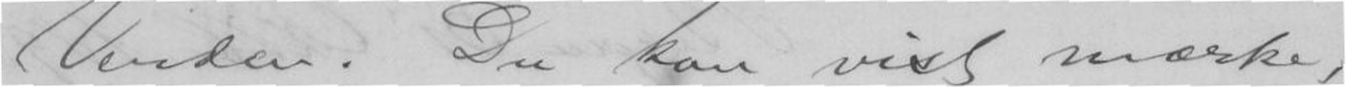Verden. Du kan vist mærke,
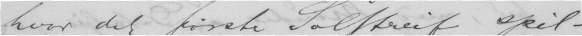hvor det første Solstrief spil-
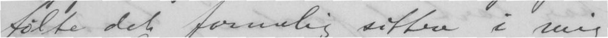følte det formelig sittre i mig
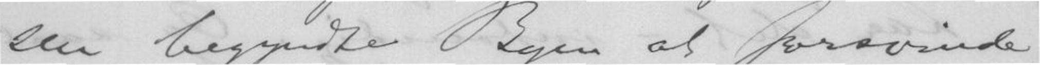sen begyndte Byen at forsvinde
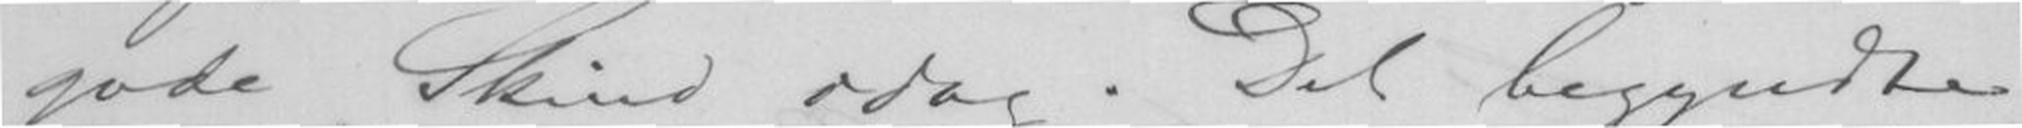gode Skin idag. Det begyndte
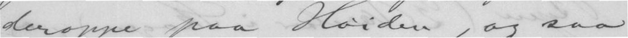deroppe paa Høiden, og saa
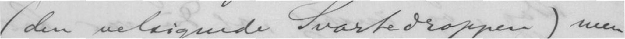(den velsignede Svartedroppen) men
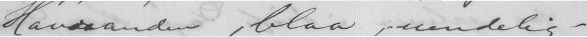Havranden, blaa, uendelig -
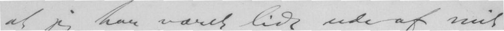at jeg har været lidt ude af mit
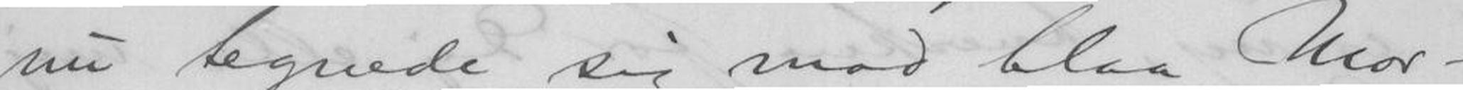nu tegnede sig mod blaa Mor-
